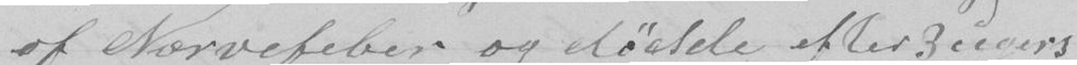of Nærvefeber og dødde efter 3 ugers
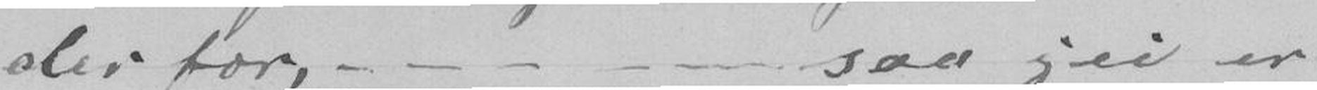der for, - - - - - - saa jei er
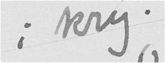i krig.
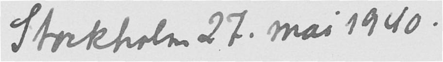Stockholm 27. mai 1940.
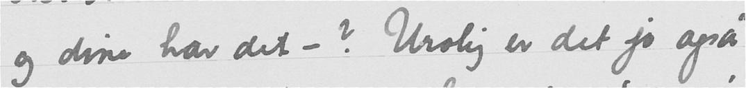og dine har det - ? Urolig er det jo også
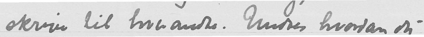skrive til hverandre. Undres hvordan du
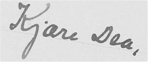Kjære Dea,
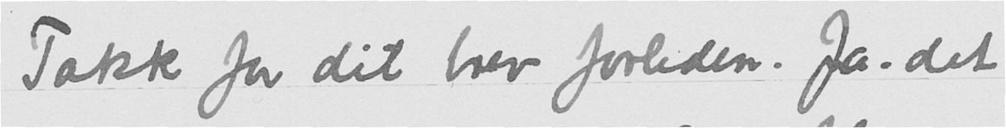Takk for dit brev forleden. Ja - det
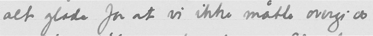alt glade for at vi ikke måtte overgi os
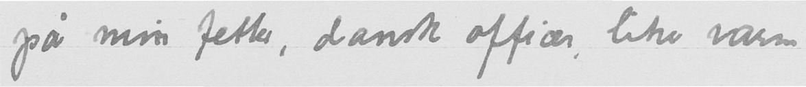på min fetter, dansk officer like varm
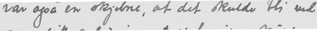var også en skjebne, at det skulde bli ved
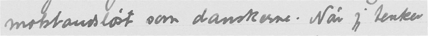motstandsløst som danskerne. Når jeg tenker
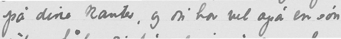på dine kanter, og du har vel også en søn
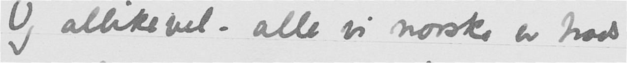Og allikevel - alle vi norske er trods
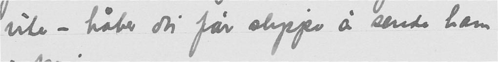ute – håber du får slippe å sende ham
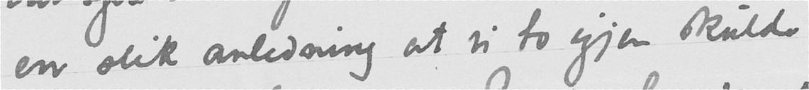en slik anledning at vi to igjen skulde
